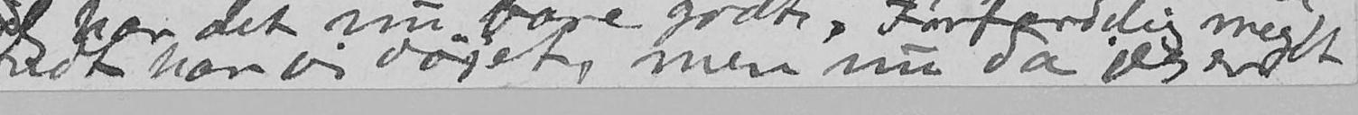ondt har jeg døjet, men nu da jeg er
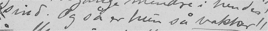sind. Og så er hun så vakker!
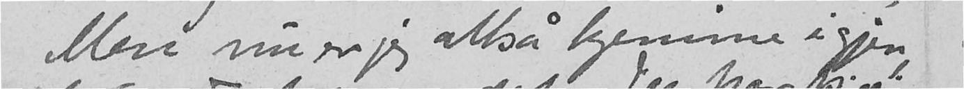Men nu er jeg altså hjemme igjen,
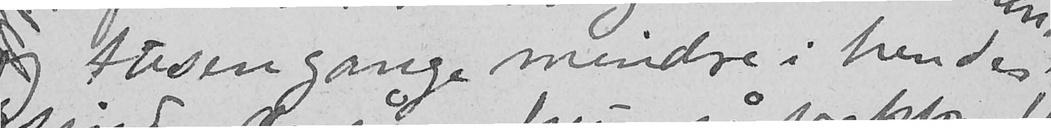og tusen gange mindre i hendes
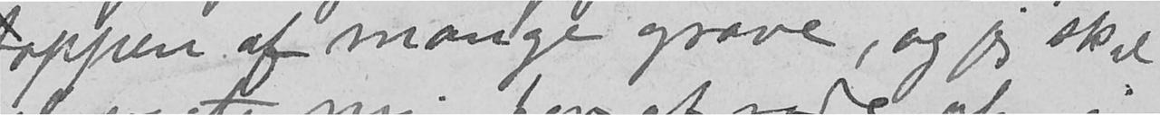toppen af mange grave, og jeg skal
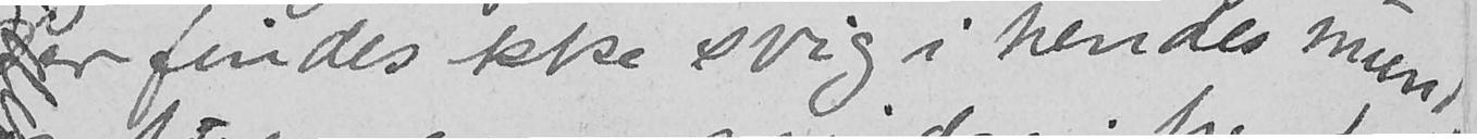Der findes ikke svig i hendes mund,
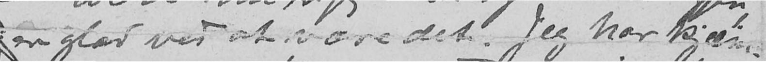og er glad ved at være det. Jeg har kjæm-
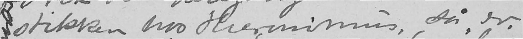i stikken hos Hieronimus, så er
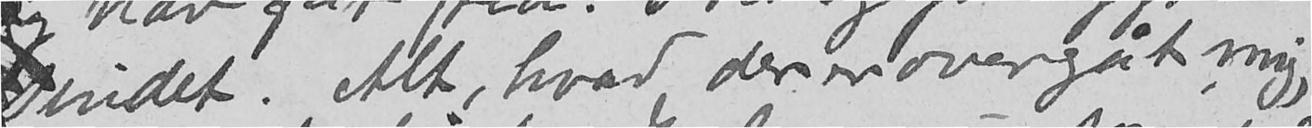sindet. Alt, hvad der er overgåt mig,
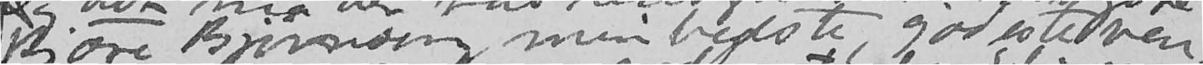kjære Bjørnson, min bedste godeste ven,
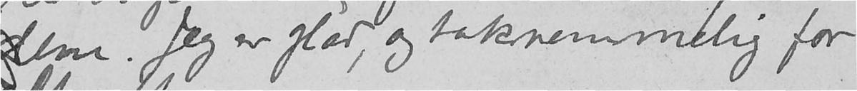dem. Jeg er glad, og taknemmelig for
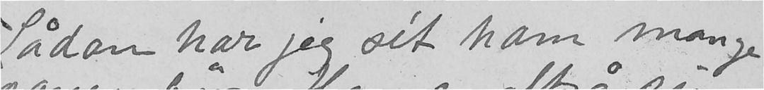Sådan har jeg sét ham mange
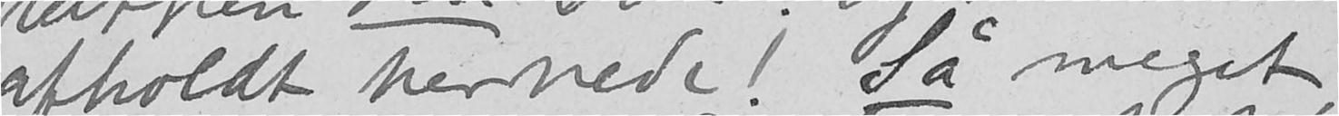afholdt hernede! Så meget,
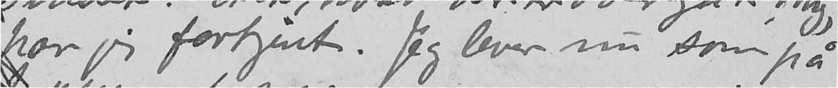har jeg fortjent. Jeg lever nu som på
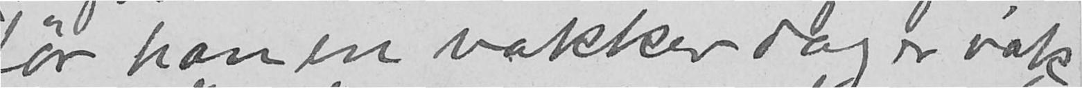før han en vakker dag er væk.
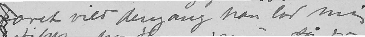faret vild dengang han lod mig
